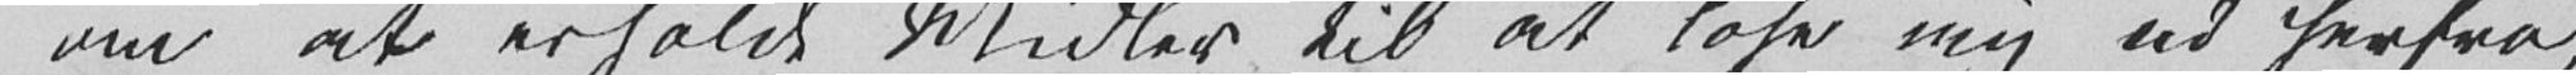om at erholde Midler til at løse mig ud herfra,
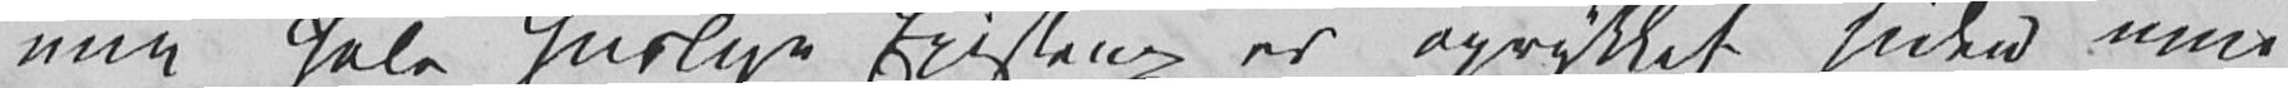min hele huslige Existenz er oprykket siden min
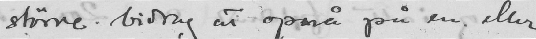større bidrag at opnå på en eller
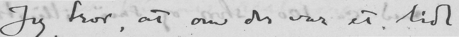Jeg tror, at om der var et lidt
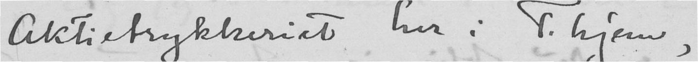Aktietrykkeriet her i T.hjem,
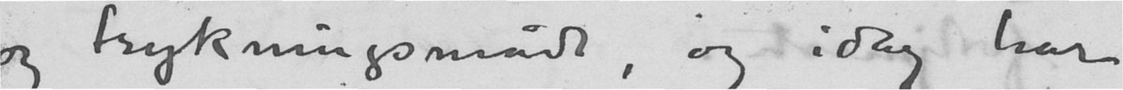og trykningsmåde, og idag har
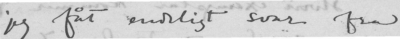jeg fåt endeligt svar fra
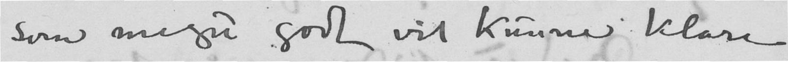som meget godt vil kunne klare
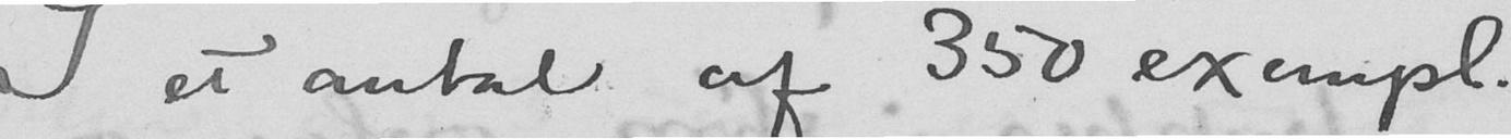I et antal af 350 exempl.
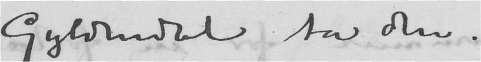Gyldendal ta den.
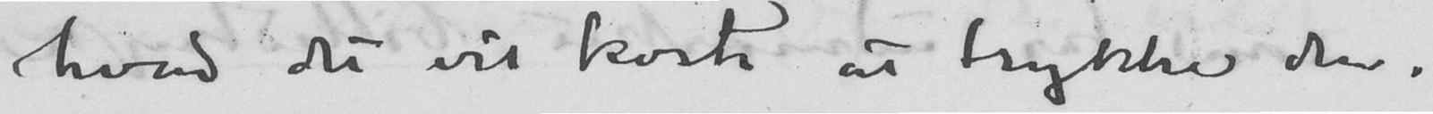hvad det vil koste at trykke den.
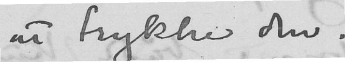at trykkde den.
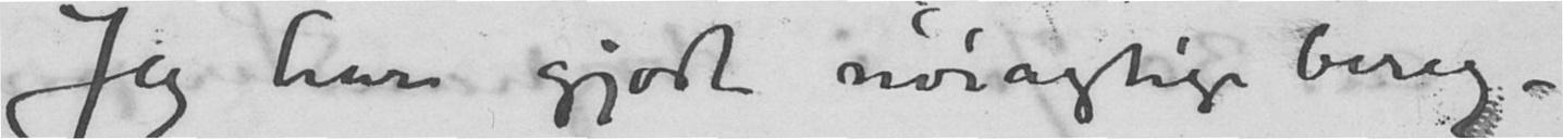Jeg har gjort nøiagtige bereg-
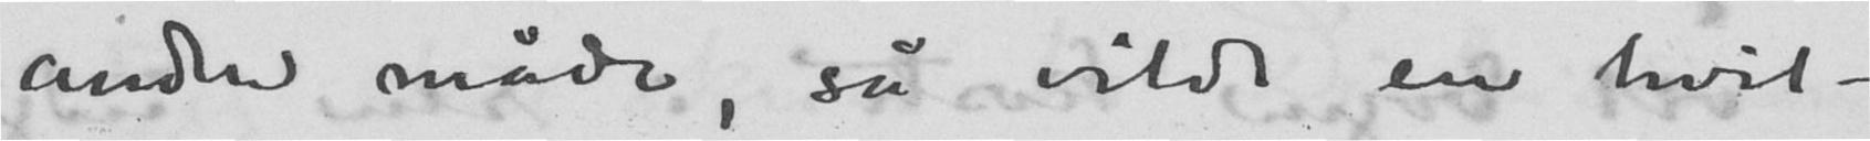anden måde, så vilde en hvil-
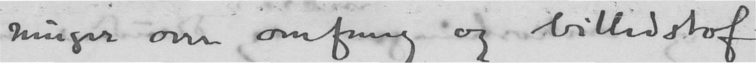ninger om omfang og billedstof
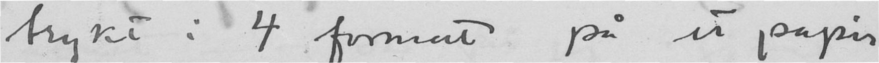trykt i 4 format på et papir
